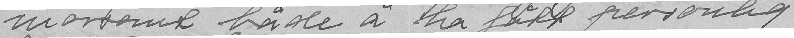morsomt både å ha fått personlig
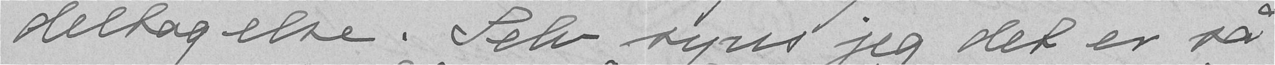deltagelse. Selv syns jeg det er så
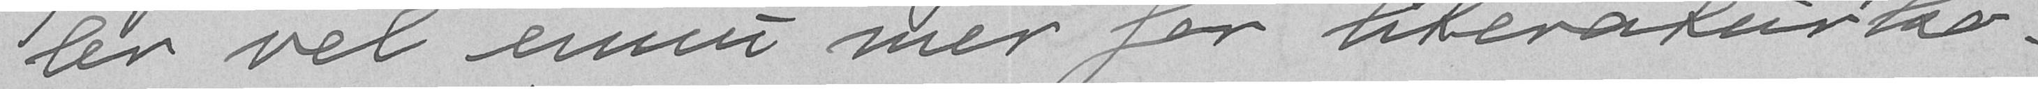ler vel ennu mer for literaturko-
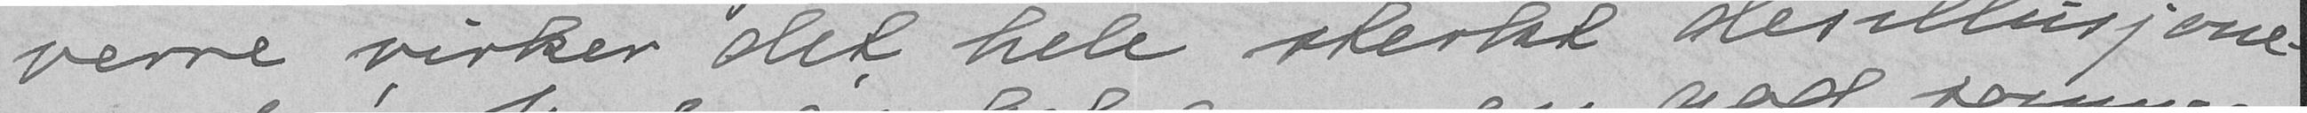verre virker det hele sterkt desillesjone-
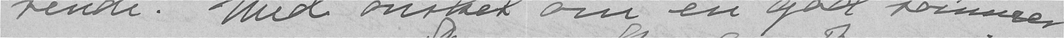rende. Med ønsket om en god sommer
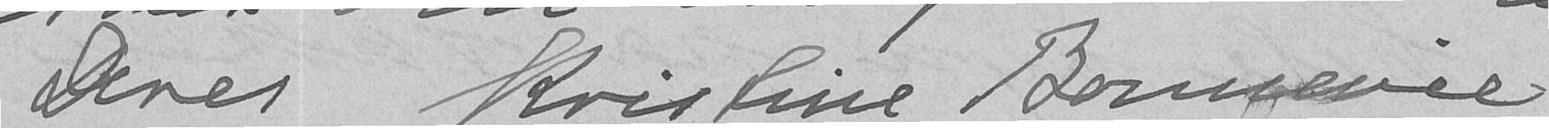Deres Kristine Bonnevie
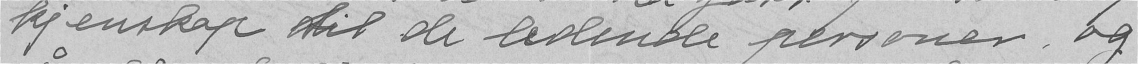kjenskap dit de ledende personer, og
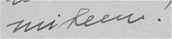miteen!
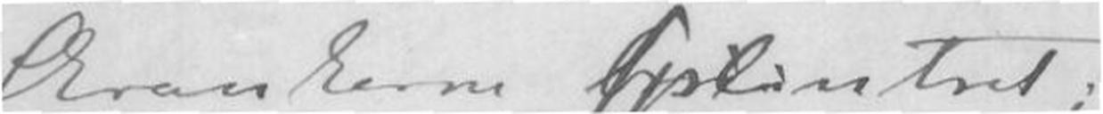Skrankerne splintret;
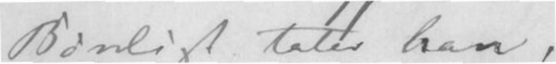Bønligt taler han,
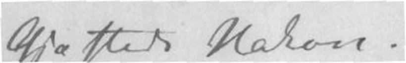Gjæsteds Hakon.
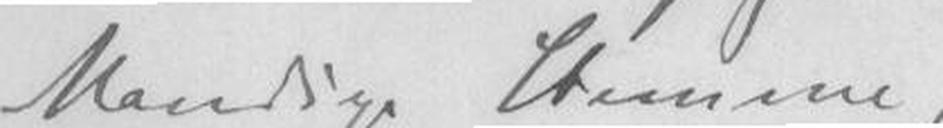Mandige Stemme,
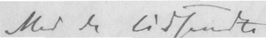Med de Uidsendte
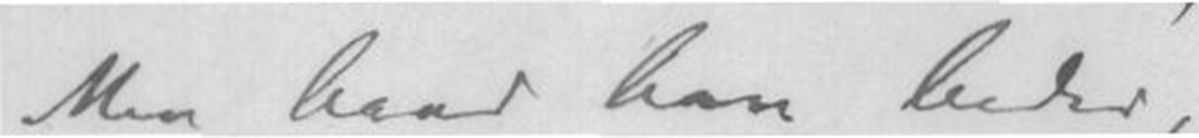Men hvad han beder,
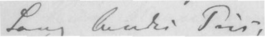Sang burde Pris,
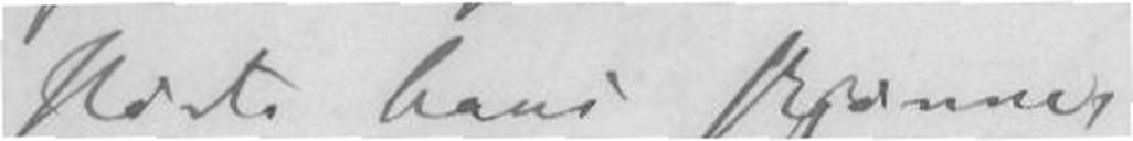Hørte hans skjønne,
